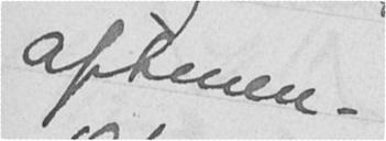aftenen.
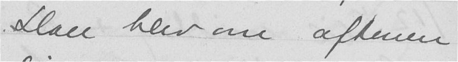Han blev om aftenen
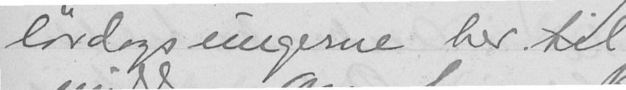lørdagsungerne her til
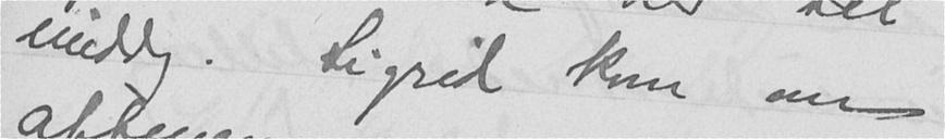middag. Sigrid kom om
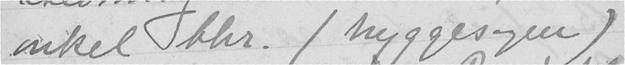onkel Chr. (byggesagen)
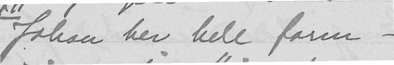Johan her hele form -
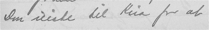Don reiste til Kria for at
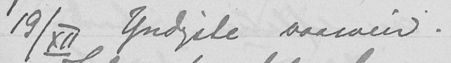19/XII Yndigste vaarveir.
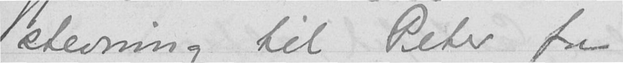stevning til Peter fra
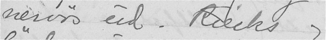nervøs ud. Riecks og
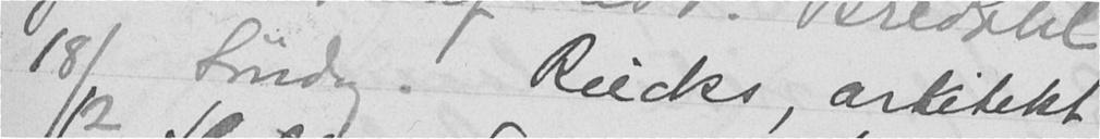18/12 Søndag. Riecks, arkitekt
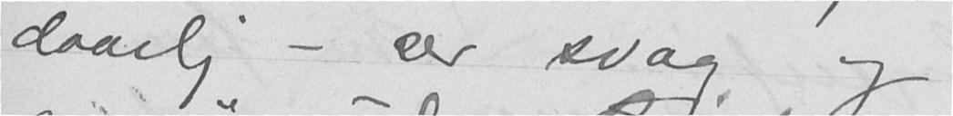daarlig - ser svag og
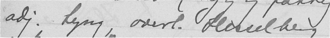adj. Lyng, overl. Hesselberg,
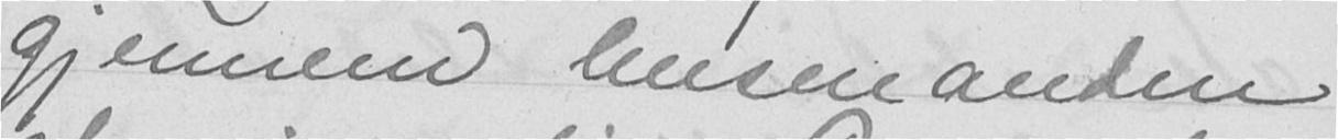gjennem lensmanden
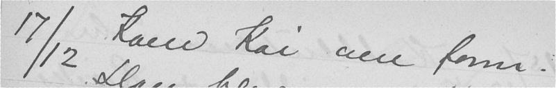17/12 Kom Kai om form.
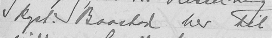kapt. Baastad her til
